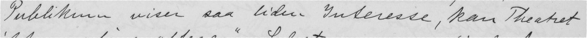Publikum viser saa liden Interesse, kan Theatret,
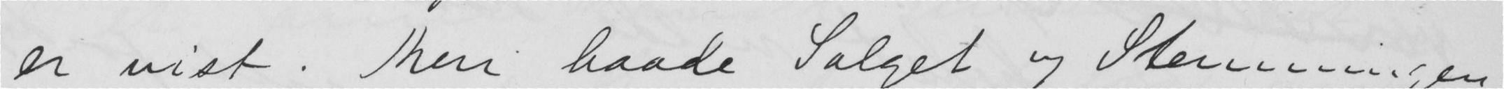er vist. Men baade Salget og Stemningen
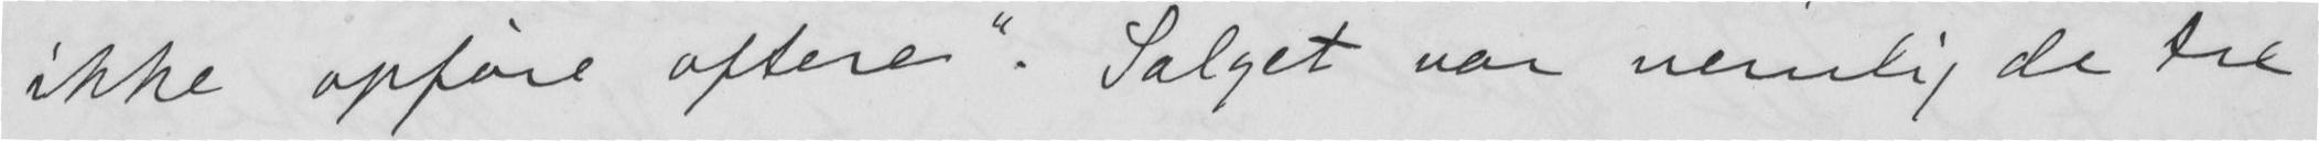ikke opføre oftere". Salget var nemlig de tre
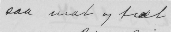saa mat og træt
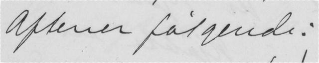Aftener følgende:
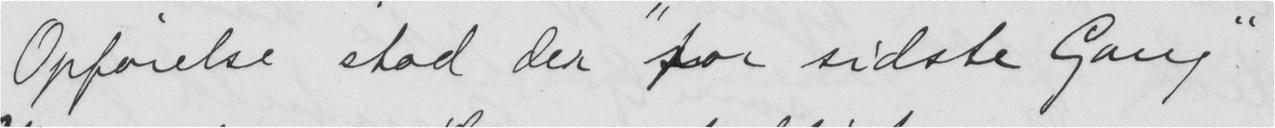Opførelse stod der "for sidste Gang".
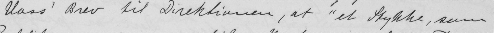Voss' Brev til Direktionen, at "et Stykke, som
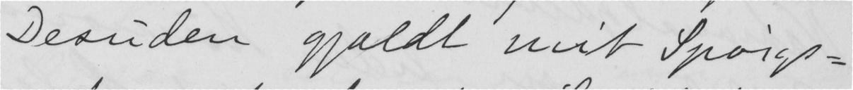Desuden gjaldt mit Spørgs-
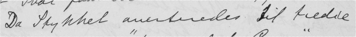Da Stykket averteredes til tredie
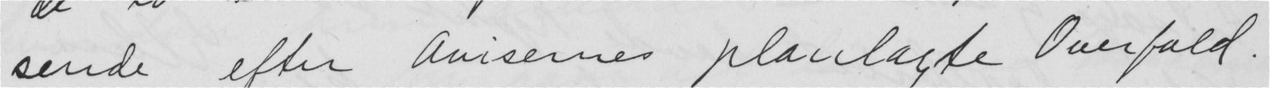sende efter Avisernes planlagte Overfald.
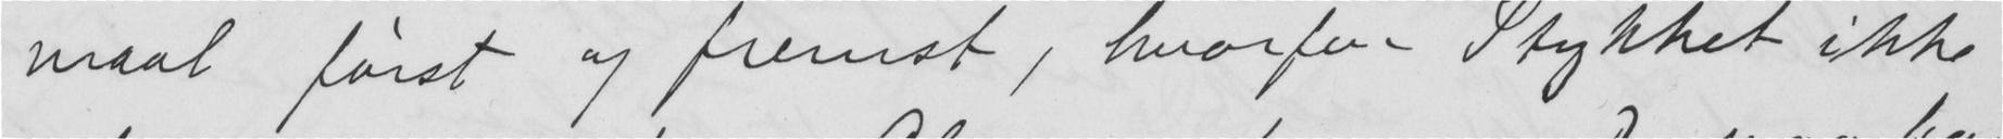maal først og fremst, hvorfor Stykket ikke
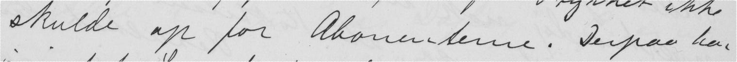skulde op for Abonenterne. Derpaa har
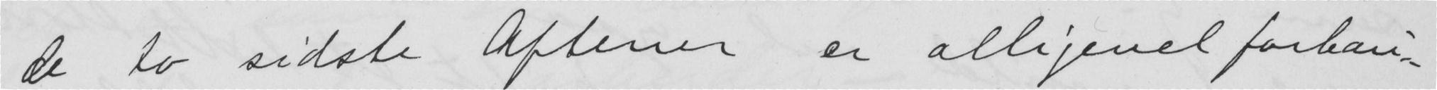de to sidste Aftener er alligevel forbau-
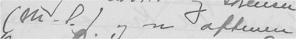(M. S.) og om aftenen
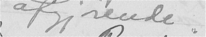afgjørende.
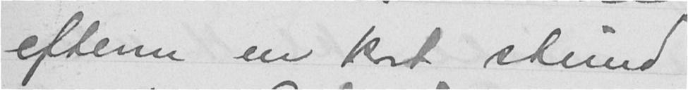efterm en kort stund
